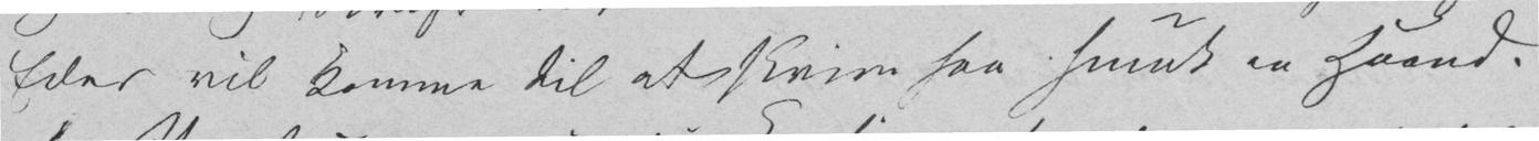Eder vil komme til at skrive saa smuk en Haand.
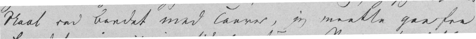Skaal ved Bordet med Taarer, jeg maatte gaa fra
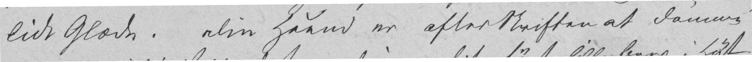lidt Glæde. Din Haand er efter Skriften at dømme
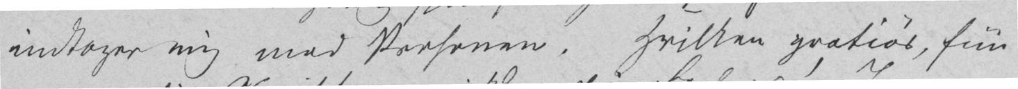indtager mig mod Personen. Hvilken gratiøs, fiin
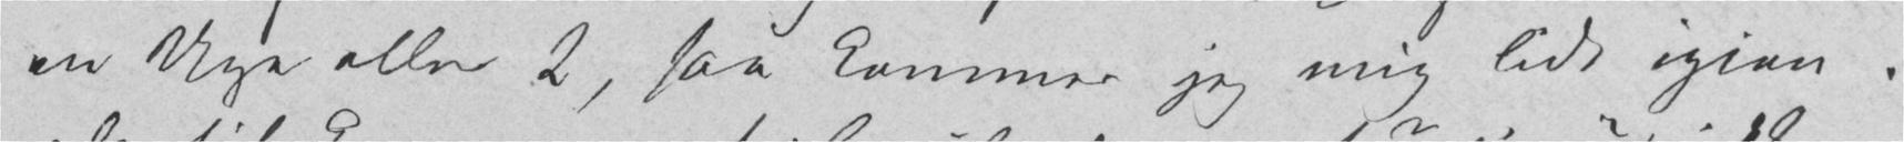en Uge eller 2, saa kommer jeg mig lidt igjen.
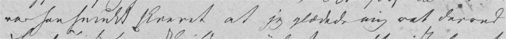var saa smukt skrevet at jeg glædede mig ret derved.
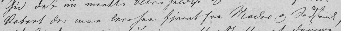Robert der maa leve saa fjernt fra Moder og Sødskende,
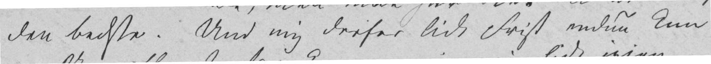den bedste. Und mig derfor lidt Frist endnu kun
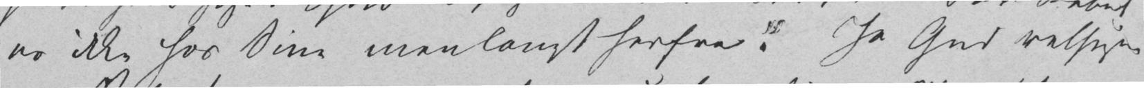er ikke hos Sine men langt herfra.» Ja Gud velsigne
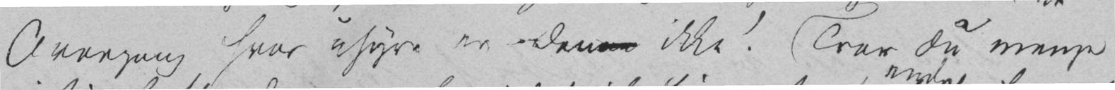Overgang hvor uhyre er denne ikke! Tror Du mange
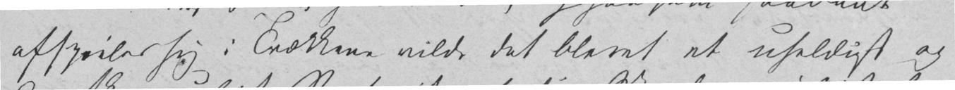afspeiler sig i Trækkene vilde det blevet et uheldigt og
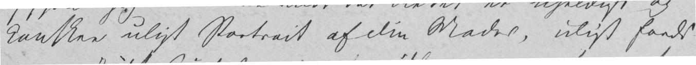kanskee uligt Portrait af Din Moder, uligt fordi
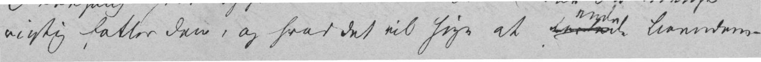rigtig fatter den, og hvad det vil sige at forlade Barndommens
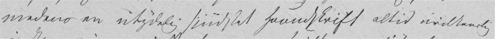medens en utydelig sjudsket Haandskrift altid uvilkaarlig
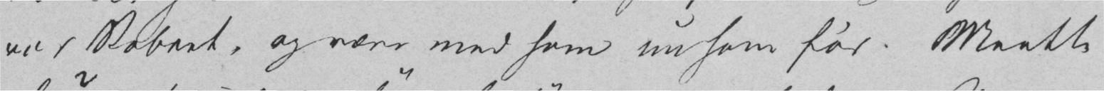vor Robert, og være med ham nu som før. Maatte
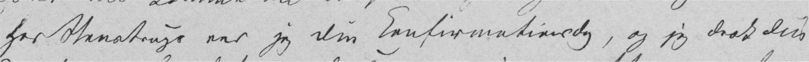Hos Stenstrups var jeg Din Confirmationsdag, og jeg drak Din
